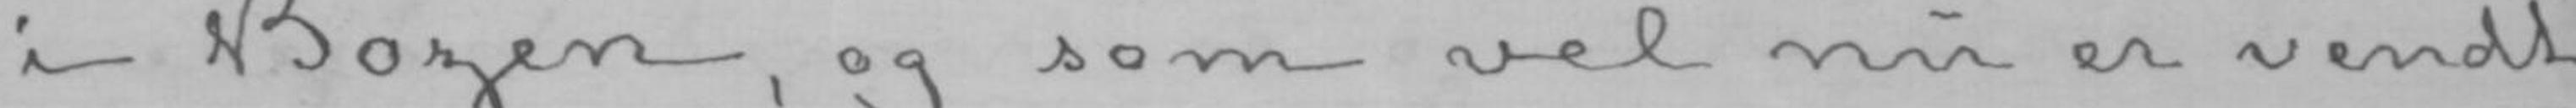i Bozen, og som vel nu er vendt
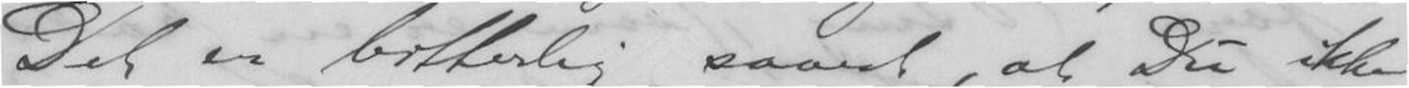Det er bitterlig saart, at Du ikke
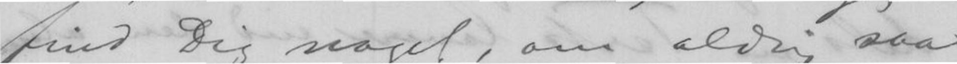find Dig noget, om aldrig saa
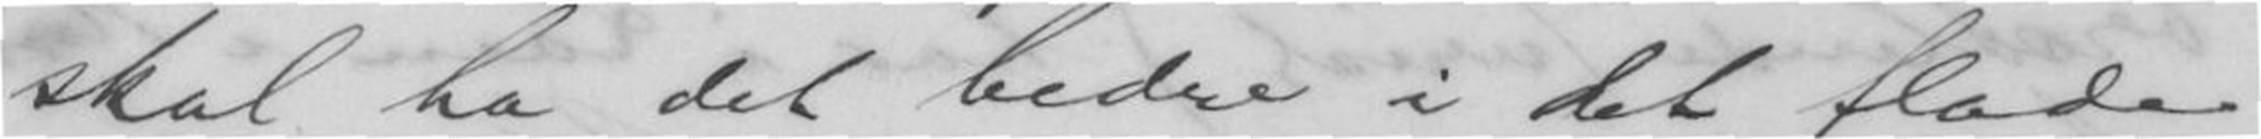skal ha det bedre i det flade
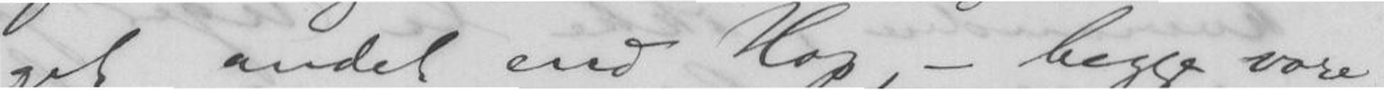get andet end Hop, - begge vore
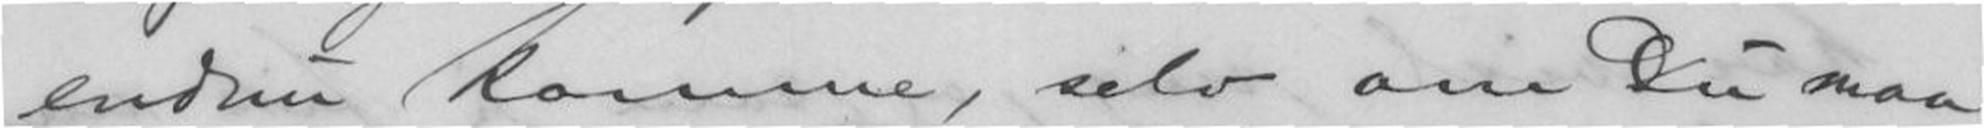endnu komme, selv om Du maa
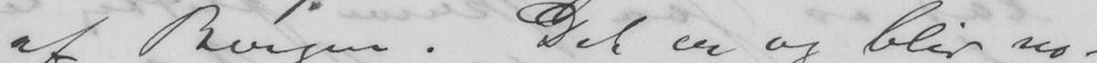af Bergen. Det er og blir no-
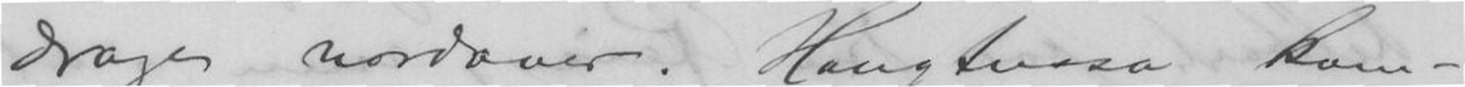drage nordover. Haugtussa kom-
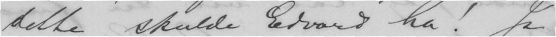dette skulde Edvard ha! Ja,
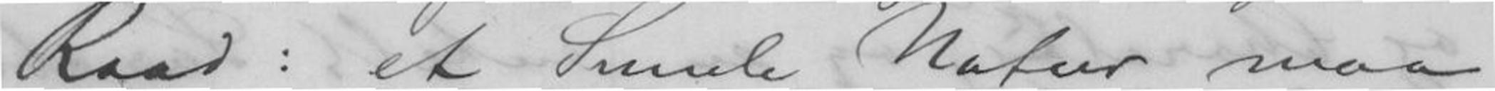Raad: et Smule Natur maa
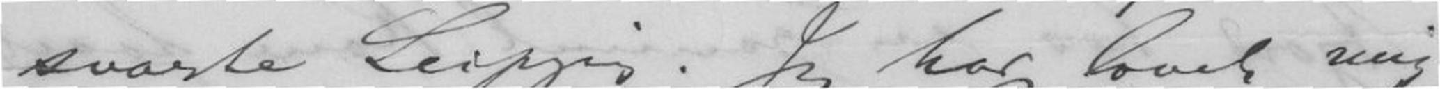svarte Leipzig. Jeg har lovet mig
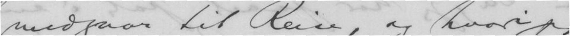medgaar til Reise, og hvori jeg
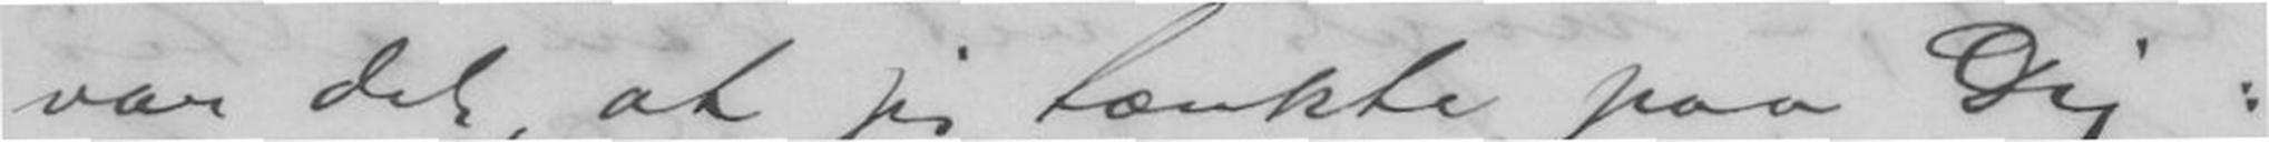var det, at jeg tænkte paa Dig:
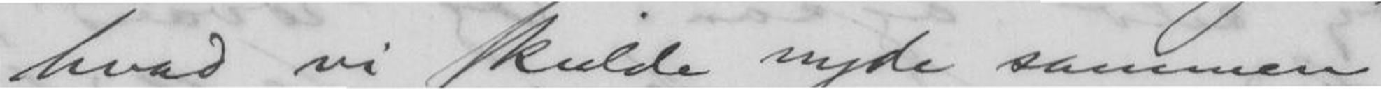hvad vi skulde nyde sammen
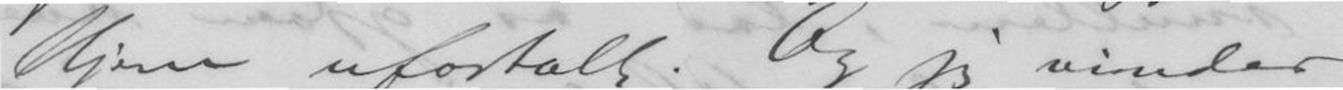Hjem ufortalt. Og jeg vinder
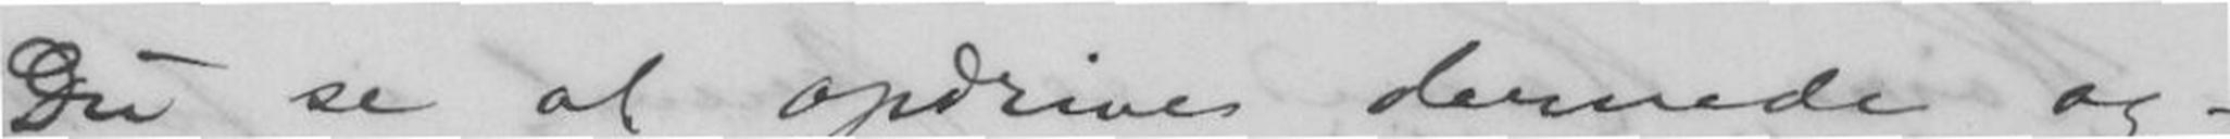Du se at opdrive dernede og-
